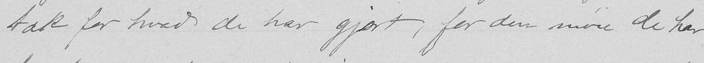tak for hvad de har gjort, for den møie de har
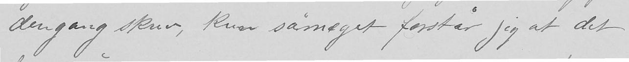dengang skrev, kun såmeget forstår jeg at det
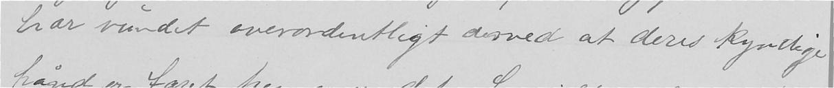har vundet overordentligt derved at deres kyndige
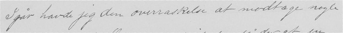Igår havde jeg den overraskelse at modtage nogle
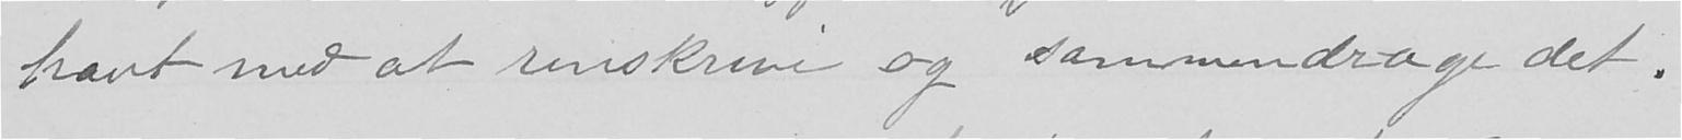havt med at renskrive og sammendrage det.
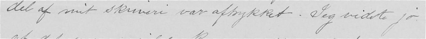del af mit skriveri var aftrykket. Jeg vidste jo
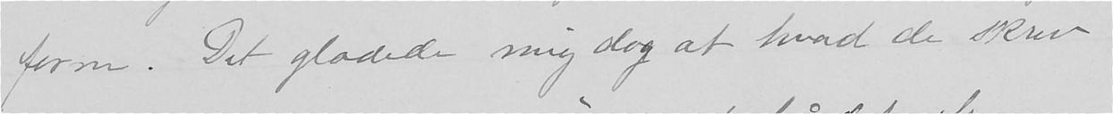form. Det glædede mig dog at hvad de skrev
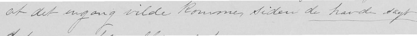at det engang vilde komme, siden de havde sagt
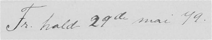Fr. hald 29de mai 79.
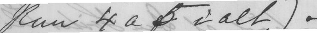kun 4 a 5 i alt) _____
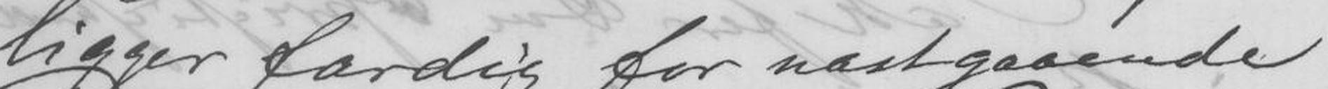ligger færdig for næstgaaende
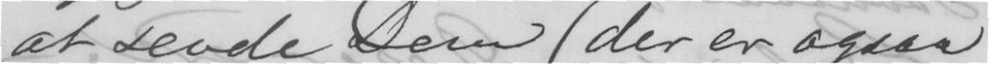at sende Dem (der er ogsaa
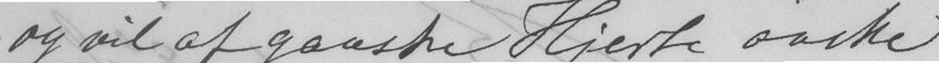og vil af ganske Hjerte ønske
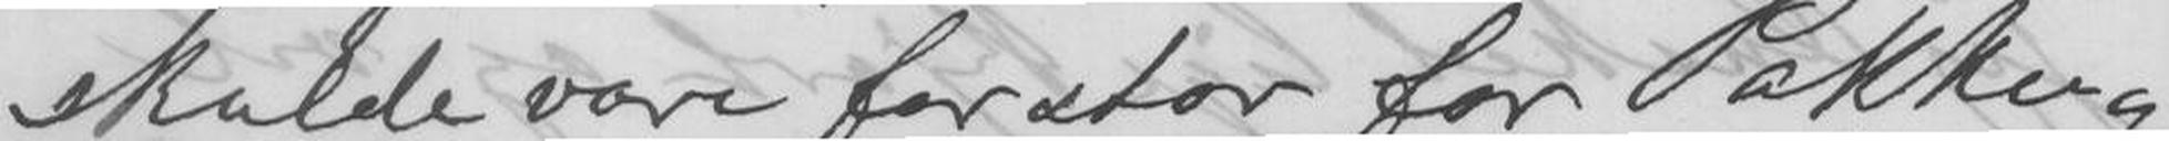skulde være for stor for Pakke-
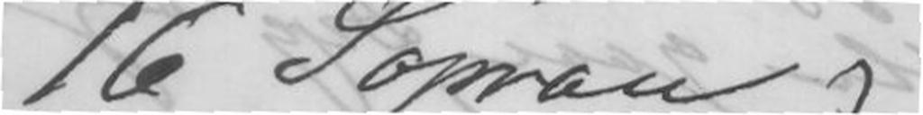16 Sopran
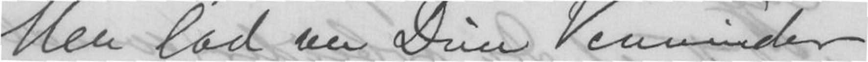Men lad nu Dine Venninder
